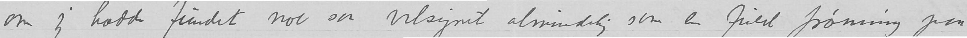om jeg hadde fundet noe saa velsignet almindelig som en fuld frøning paa
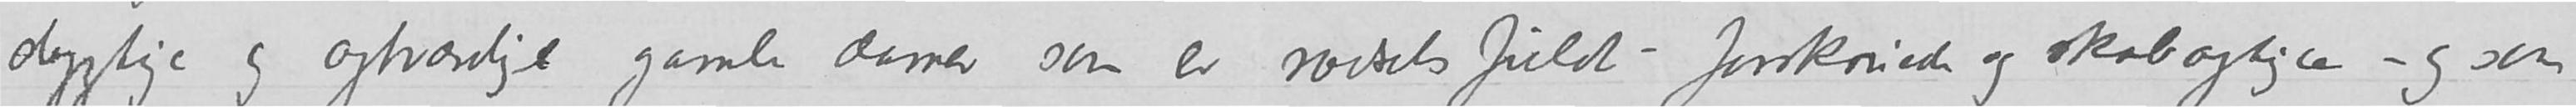dygtige og agtværdige gamle damer som er rædselsfuld-forskruede og skabagtige –- og som
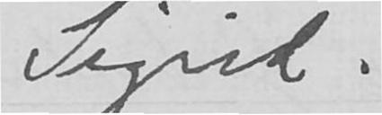Sigrid.
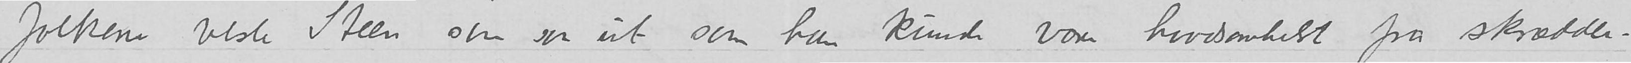vesle Steen som ser ut som han kunde være hvadsomhelst fra skrædder
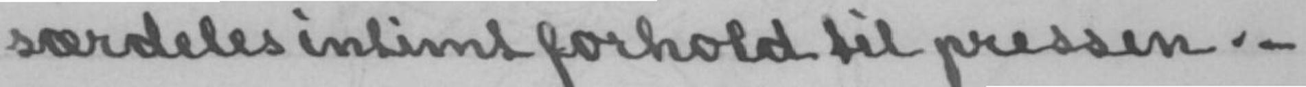særdeles intimt forhold til pressen.-
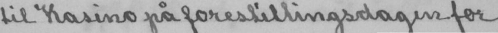til Kasino på forestillingsdagen for
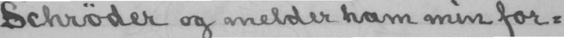Schrøder og melder ham min for-
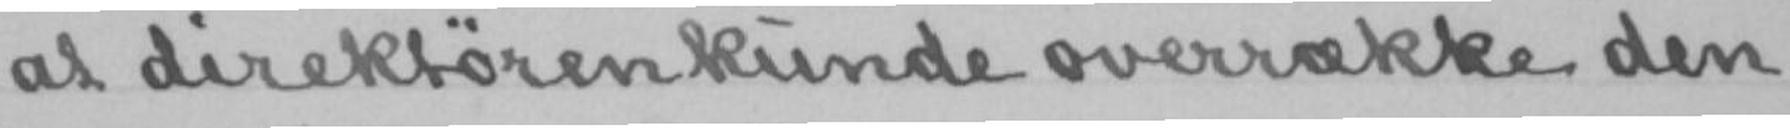at direktøren kunde overrække den
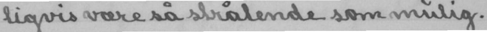ligvis være så strålende som mulig.
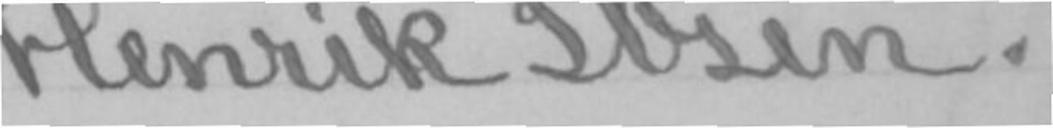Henrik Ibsen.
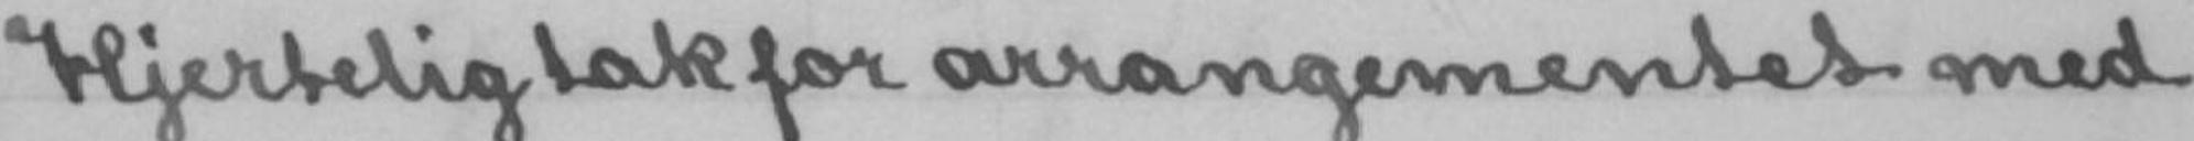Hjertelig tak for arrangementet med
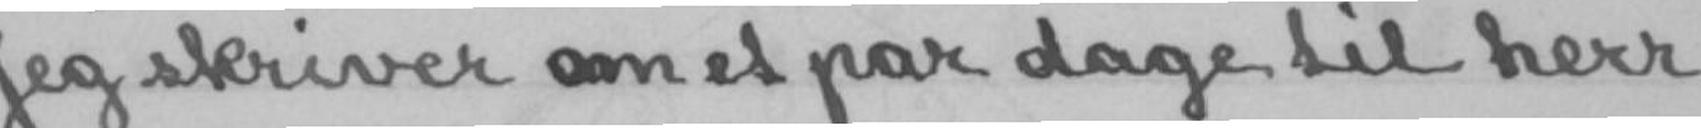Jeg skriver om et par dage til herr
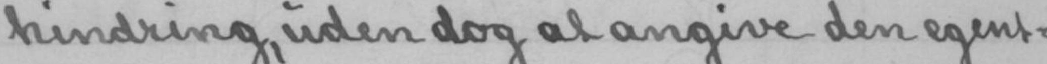hindring, uden dog at angive den egent-
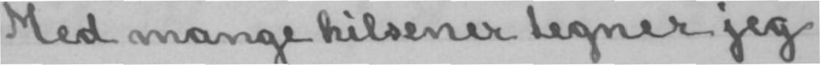Med mange hilsener tegner jeg
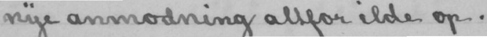nye anmodning altfor ilde op.
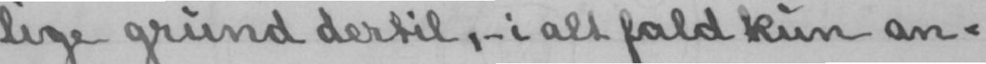lige grund dertil,- i alt fald kun an-
